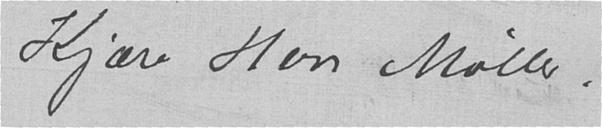Kjære Herr Møller.
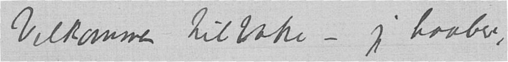Velkommen tilbake - jeg haaber
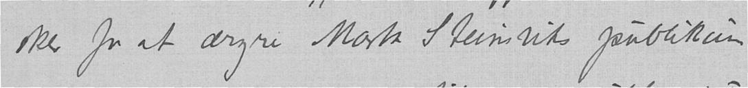sker for at ærgre Marta Steinsviks publikum
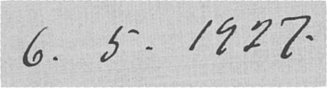6.5.1927.
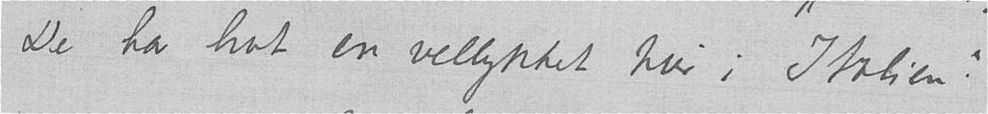De har hat en vellykket tur i Italien?
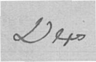Deres
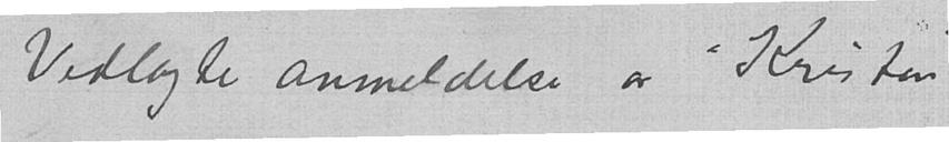Vedlagte anmeldelse av "Kristin"
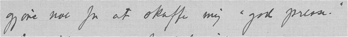gjøre noe for at skaffe mig "god presse."
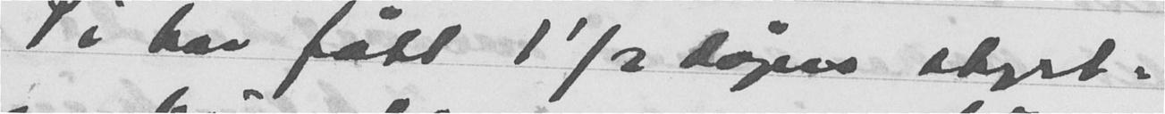Vi har fått 1 1/2 døgns styrt-
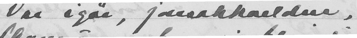Var igår, jansakkuelden,
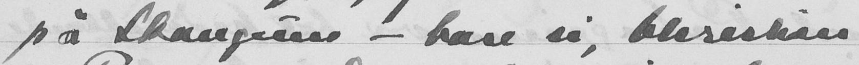på Skaugum - bare si, Claresken
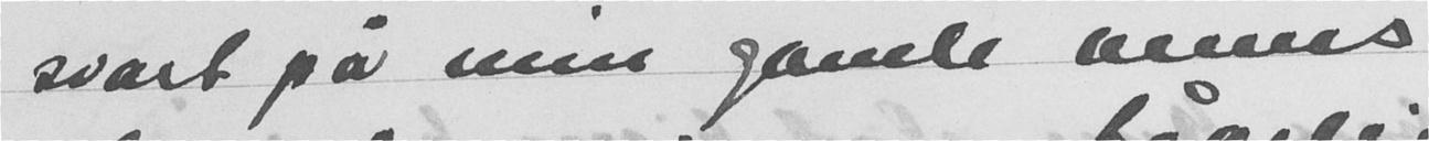svart på min gamle venns
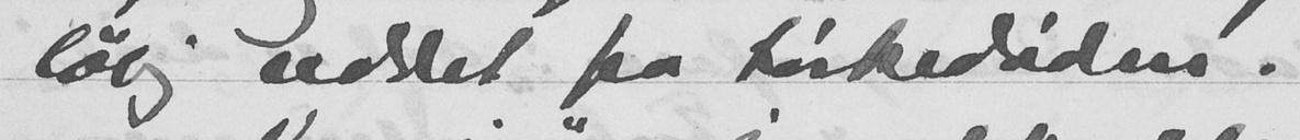løbig siddet fra tirkedadens.
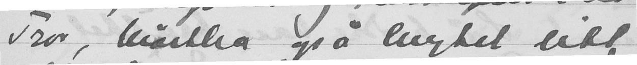Tror, Märtha også lengtet litt
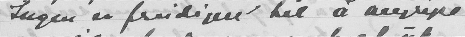Ingen er freidigere til å angripe
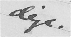dige.
